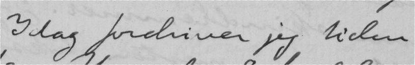Idag fordriver jeg tiden
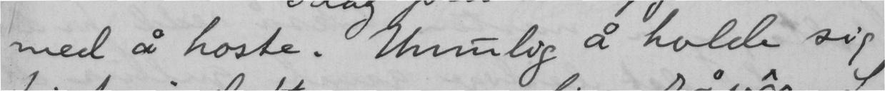med å hoste. Umulig å holde sig
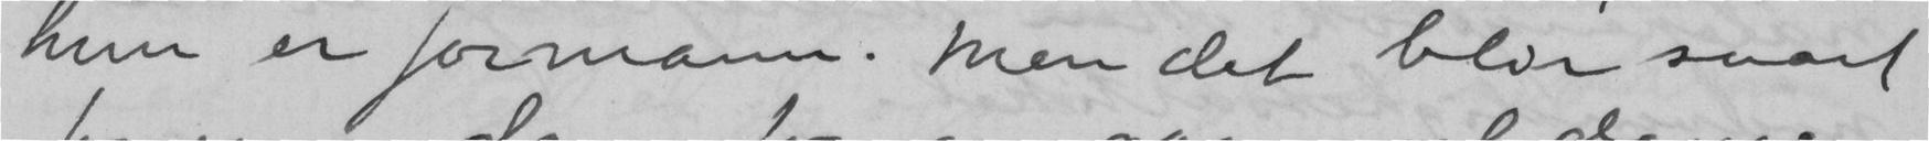hun er formann. Men det blir svært
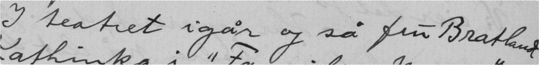I teatret igår og så fin Bratland
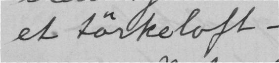et tørkeloft -
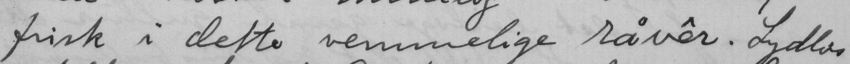frisk i dette vemmelige råvêr. Lydløs
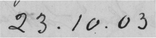23.10.03
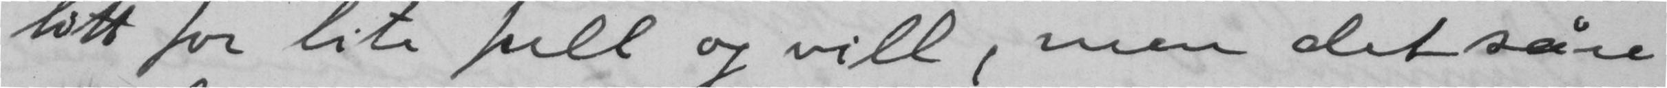litt for lite full og vill, men det såre
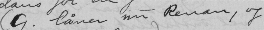G. låner nu Renan, og
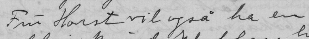Fru Horst vil også ha en
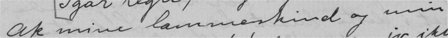Ak mine lammeskind og min
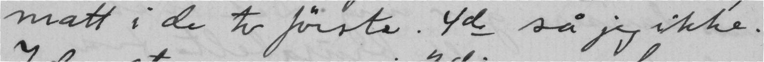matt i de to første. 4de så jeg ikke.
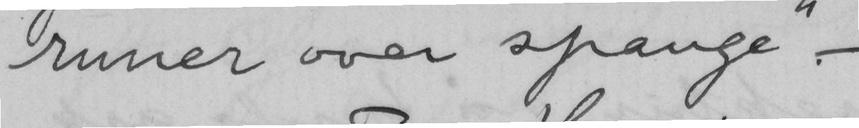runer over spange" -
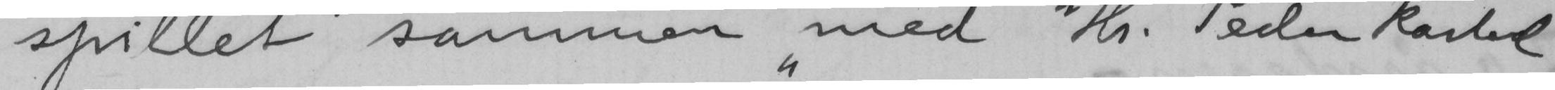spillet" sammen med "Hr. Peder kasted
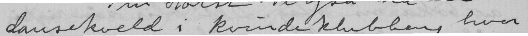dansekveld i kvindeklubben, hvor
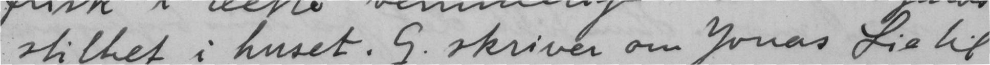stilhet i huset. G. skriver om Jonas Lie til
